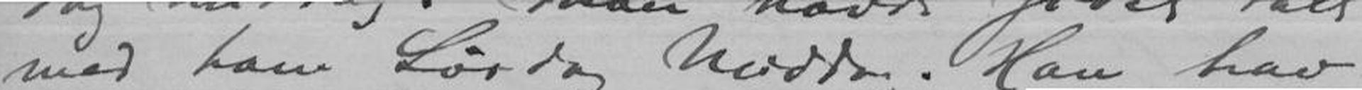med ham Lørdag Middag. Han hav-
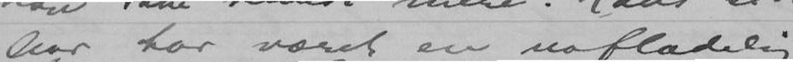Aar har været en uafladelig
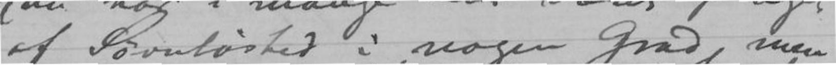af Søvnløshed i nogen Grad, men
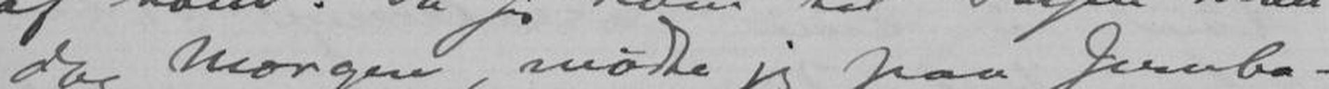dag Morgen, mødte jeg paa Jernba-
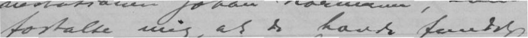fortalte mig, at de havde fundet
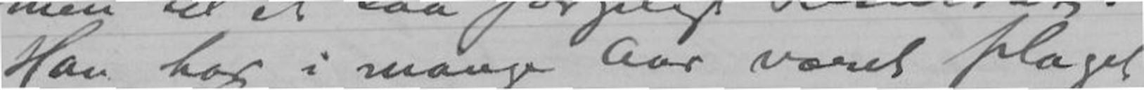Han har i mange Aar været plaget
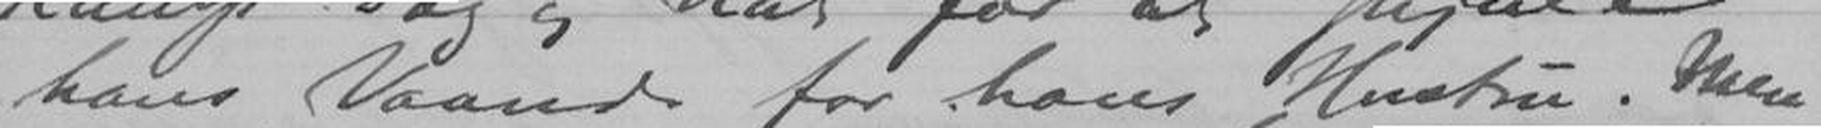hans Vaand for hans Hustru. Men
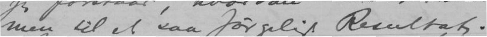men til et saa sørgelig Resultat.
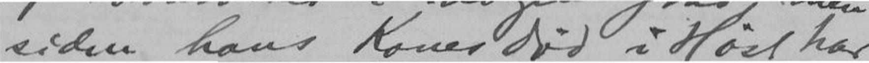siden hans Kones Død i Høst har
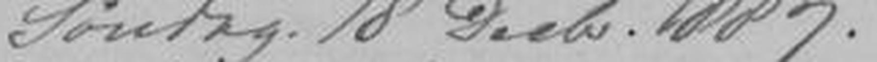Søndag 18 Decb. 1887.
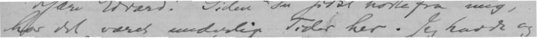har det været underlige Tider her. Jeg havde og-
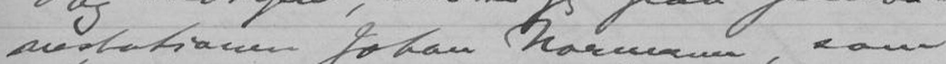nestationen Johan Normann, som
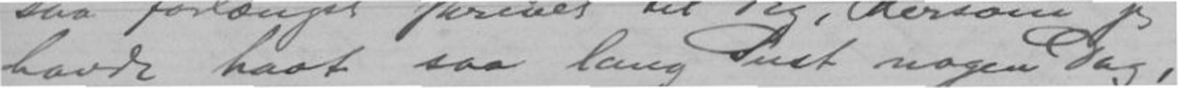havde havt saa lang Pust nogen Dag,
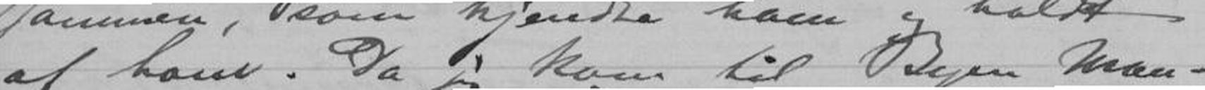af ham. Da jeg kom til Byen Man-
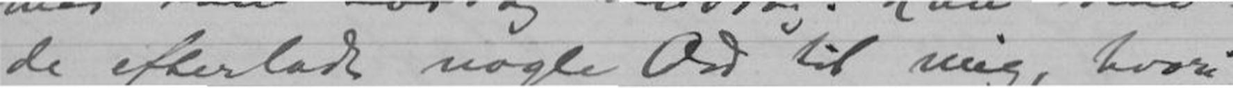de efterladt nogle Ord til mig, hvori
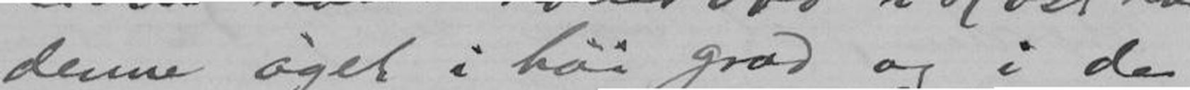denne øget i høi grad og i de
